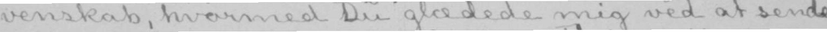venskab, hvormed Du glædede mig ved at sende
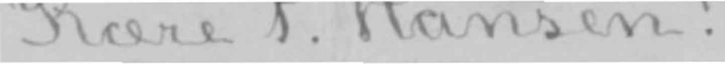Kære P. Hansen!
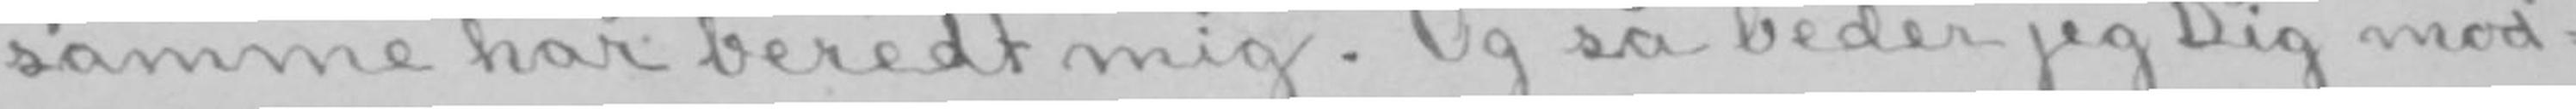samme har beredt mig. Og så beder jeg Dig mod-
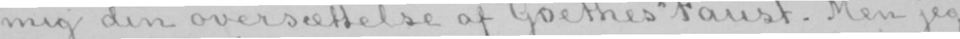mig din oversættelse af Goethes «Faust. Men jeg
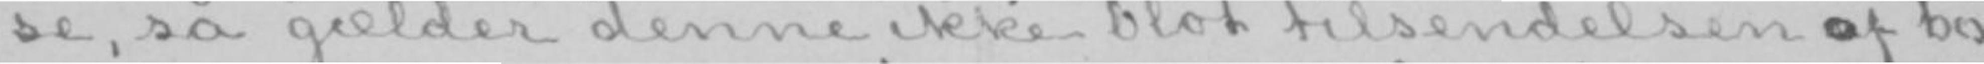se, så gælder denne ikke blot tilsendelsen af bo-
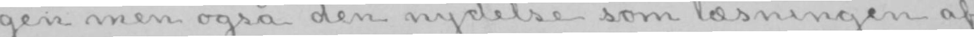gen men også den nydelse som læsningen af
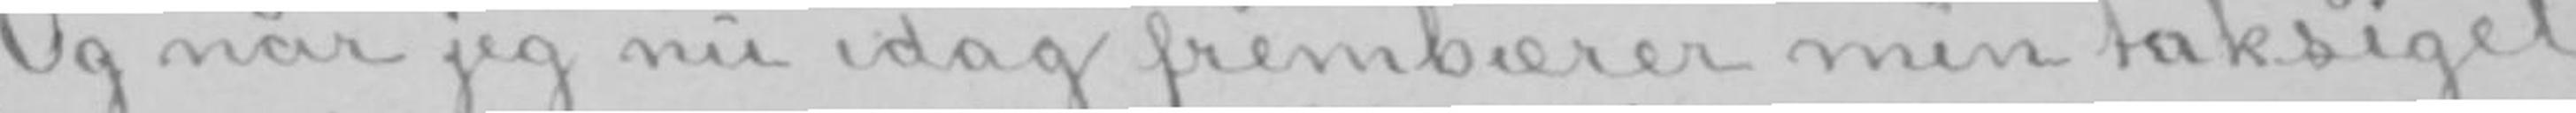Og når jeg nu idag frembærer min taksigel-
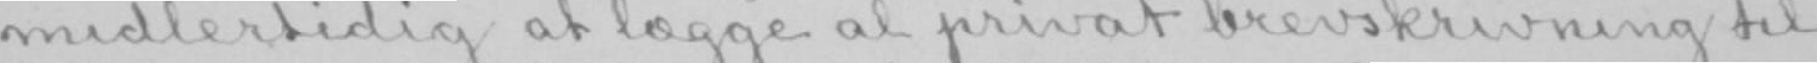midlertidig at lægge al privat brevskrivning til
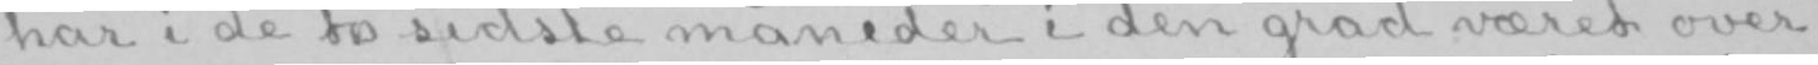har i de to sidste måneder i den grad været over-
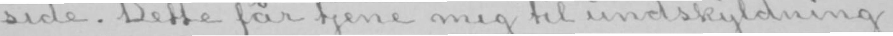side. Dette får tjene mig til undskyldning.
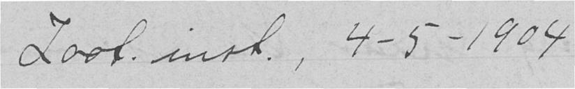Zoot. inst., 4-5-1904
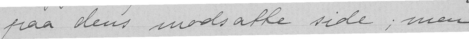paa dens modsatte side; men
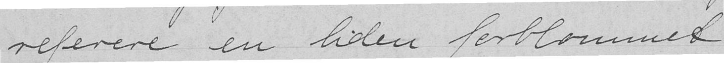referere en liden forblommet
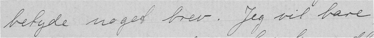betyde noget brev. Jeg vil bare
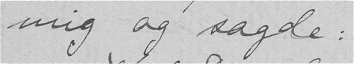mig og sagde:
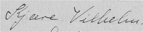Kjære Vilhelm!
